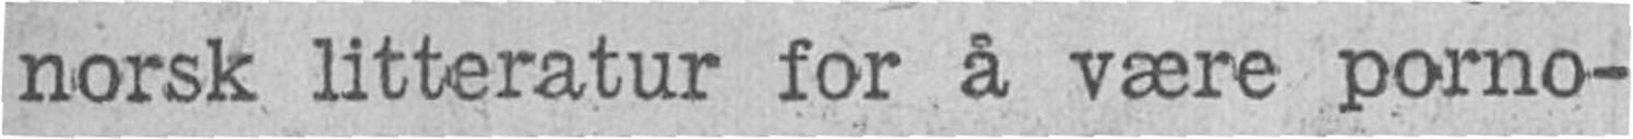norsk litteratur for å være porno-
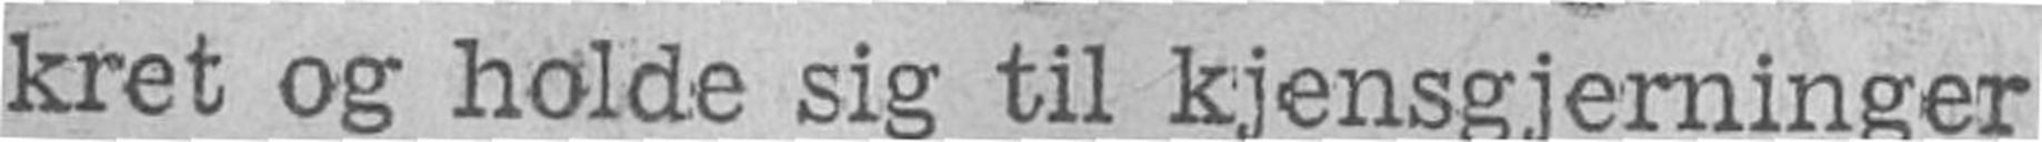kret og holde sig til kjensgjerninger
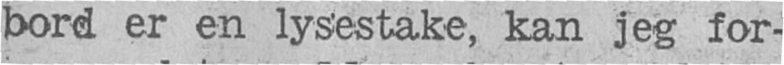bord er en lysestake, kan jeg for-
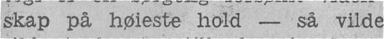skap på høieste hold - så vilde
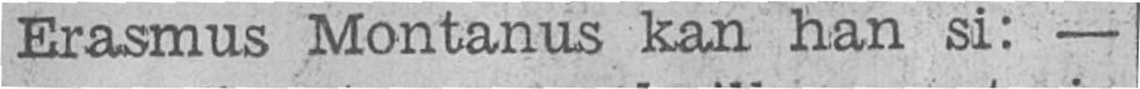Erasmus Montanus kan han si: -
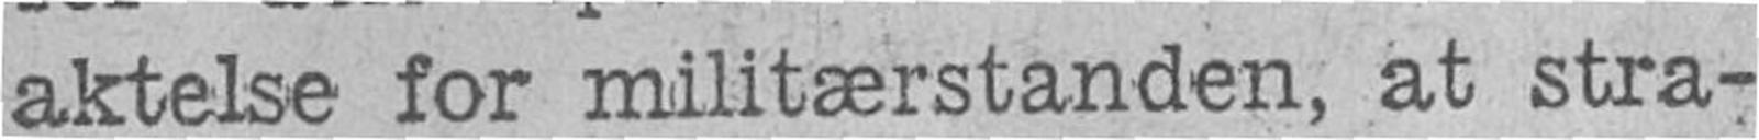aktelse for militærstanden, at stra-
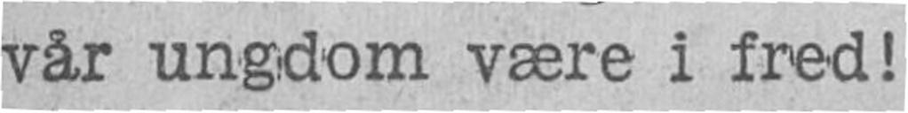vår ungdom være i fred!
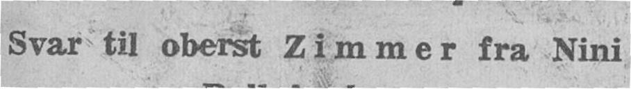Svar til oberst Zimmer fra Nini
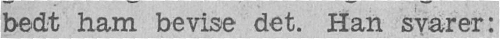bedt ham bevise det. Han svarer:
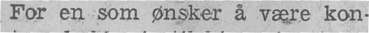For en som ønsker å være kon-
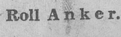Roll Anker.
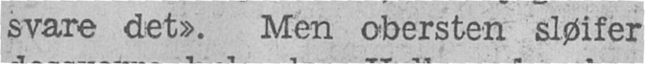svare det». Men obersten sløifer
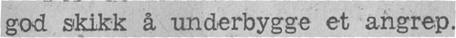god skikk å underbygge et angrep.
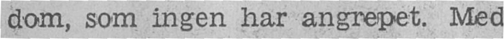dom, som ingen har angrepet. Med
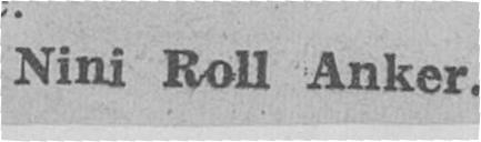Nini Roll Anker.
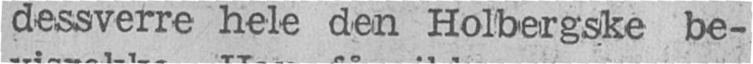dessverre hele den Holbergske be-
